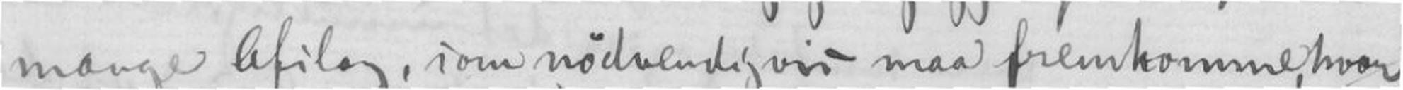mange Afslag, som nødvendigsvis maa fremkomme, hvor
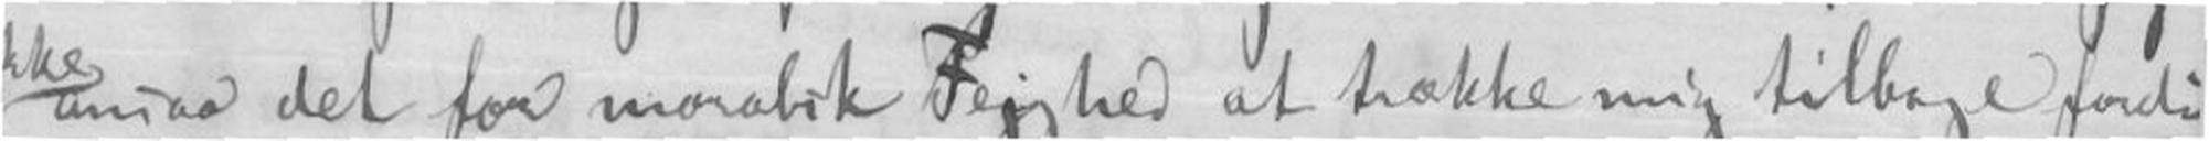ikke ansaa det for moralsk Fejghed at trække mig tilbage fordi
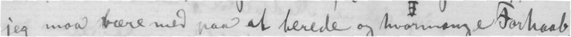jeg maa være med paa at berede og hvor mange Forhaab-
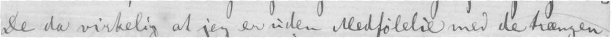De da virkelig at jeg er uden Medfølelse med de trængen
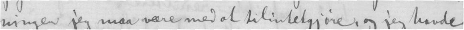ninger jeg maa være med at tilintetgjøre, og jeg havde
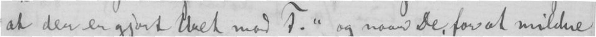"at der er gjort Uret mod F." og naar De, for at mildne
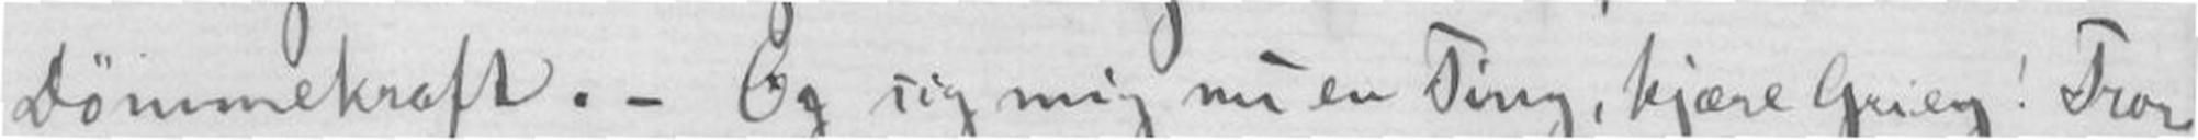Drømmekraft. - Og sig mig nu en Ting, kjære Grieg! Tror
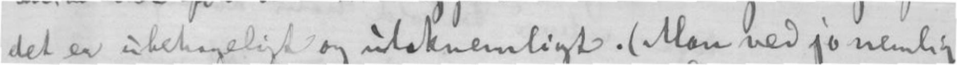det er ubehageligt og utaknemligt. (Man ved jo nemlig
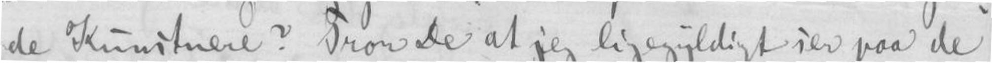de Kunstnere? Tror De at jeg ligegyldigt ser paa de
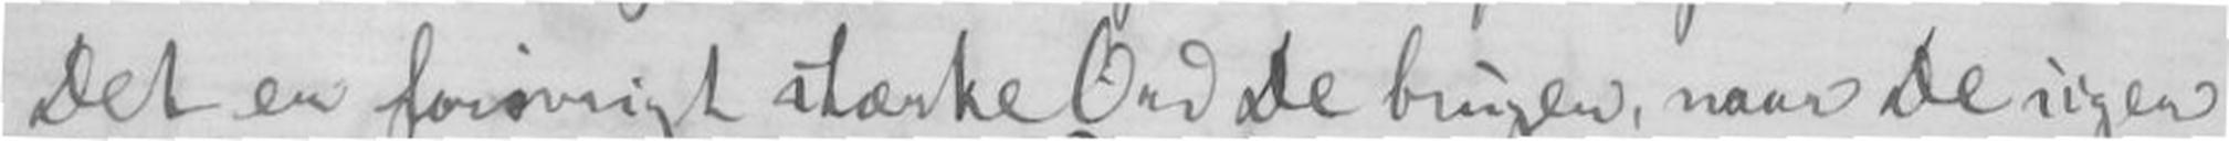Det er forøvrigt stærke Ord De bruger, naar De siger
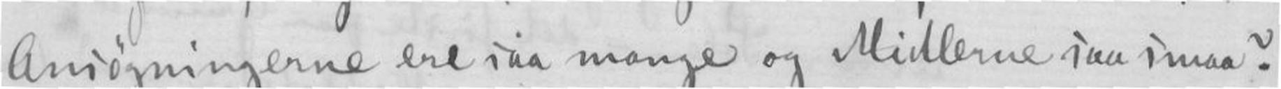Ansøgningerne ere saa mange og Midlerne saa smaa?
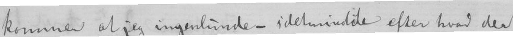kommer at jeg ingenlunde- idetmindste efter hvad der
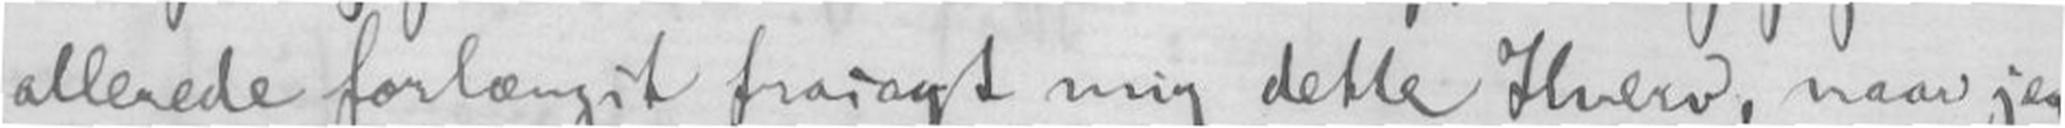allerede forlængst frasagt mig dette Hverv, naar jeg
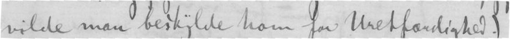vilde man beskylde ham for Uretfærdighed.)
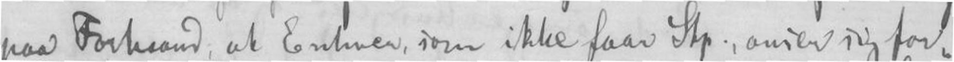paa Forhaand; at Enhver som ikke faar Stp., anser sig for-
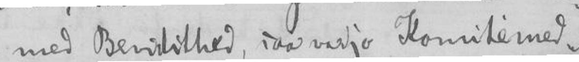med Bevidsthed, saa var jo Komièmed-
